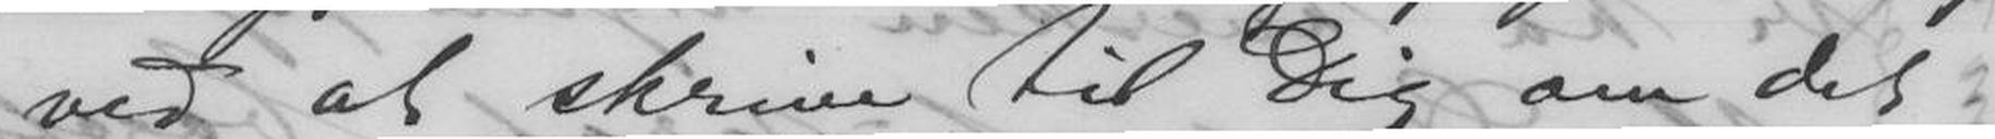ved at skrive til Dig om det
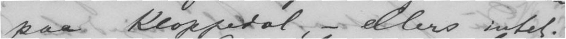paa Kloppedal, - ellers intet.
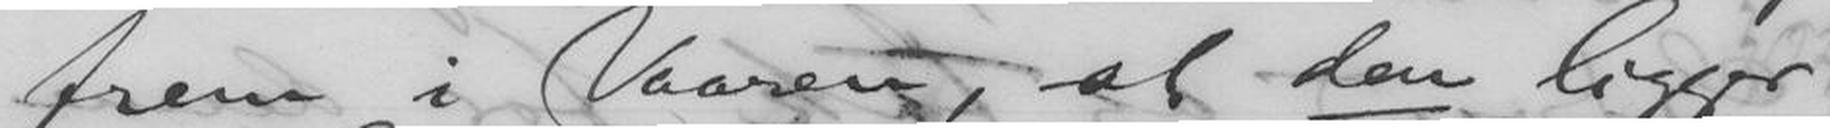frem i Vaaren, at den ligger
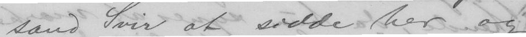sand Svir at sidde her og
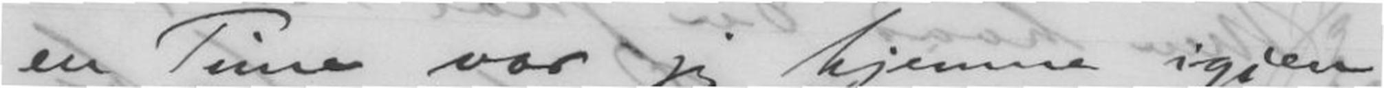en Time var jeg hjemme igjen
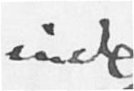inde-
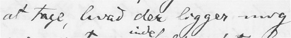at tage, hvad der ligger mig
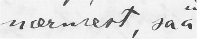nærmest, saa
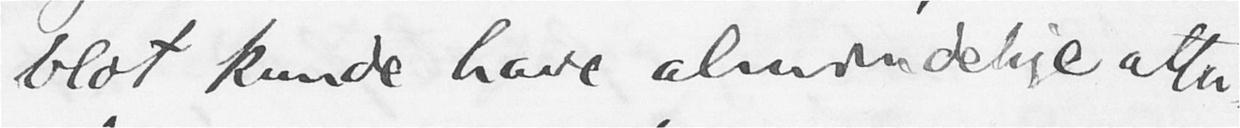blot kunde have almindelige atta-
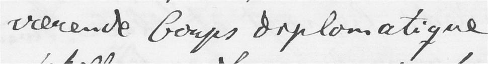værende Corps diplomatique
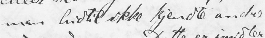man hidtil ikke kjendte andre
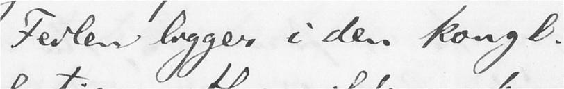Feilen ligger i den kongl.
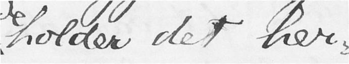holder det her-
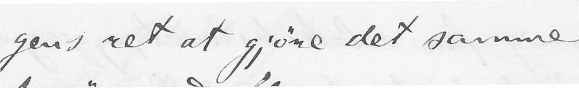gens ret at gjøre det samme
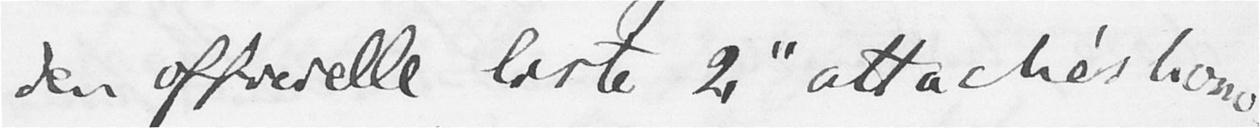den officielle liste 2 "attachés hono-
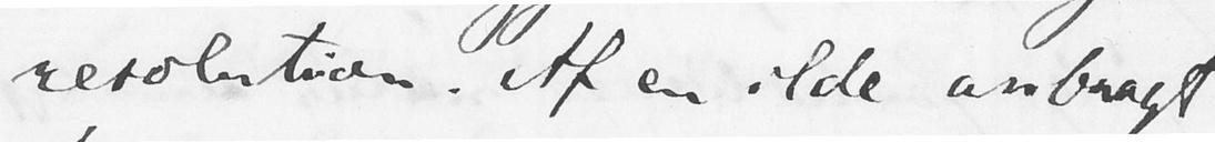resolution. Af en ilde anbragt
